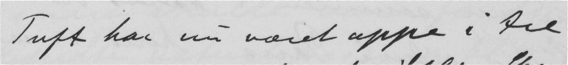Tuft har nu været oppe i tre
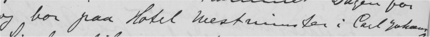og bor paa Hotel Westminster i Carl Johan
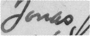Jonas
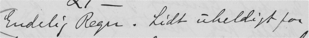Endelig Regn. Lidt uheldigt for
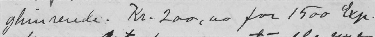glimrende. Kr. 200, uo for 1500 Exp.
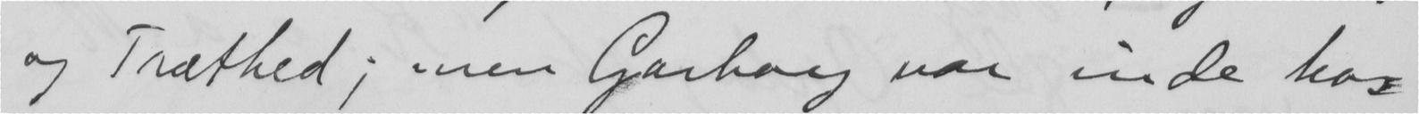og Træthed; men Garborg var inde hos
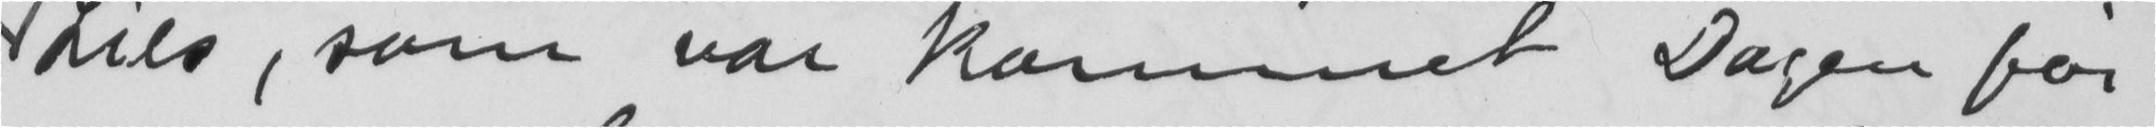Lies, som var kommet Dagen før
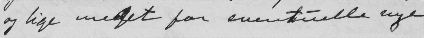og lige meget for eventuelle nye
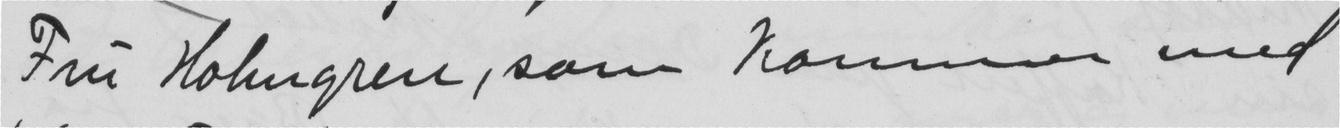Fru Holmgren, som kommer med
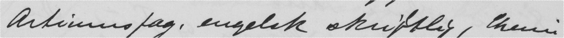Artiumsfag, engelsk skriftlig, Chemi
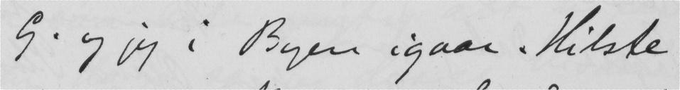G. og jeg i Byen igaar. Hilste
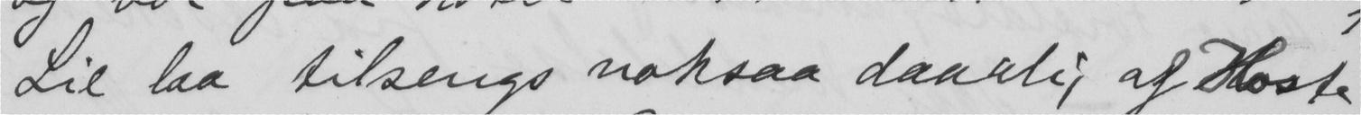Lie laa tilsengs noksaa daarlig af Hoste
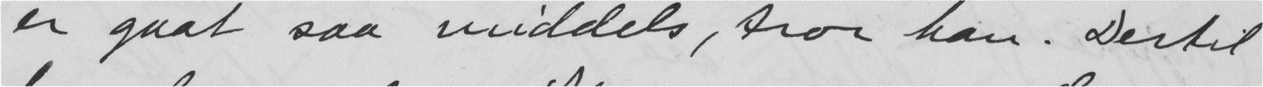er gaat saa middels, tror han. Dertil
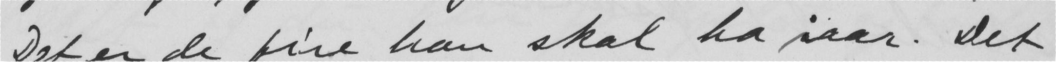Det er de fire han skal ha iaar. Det
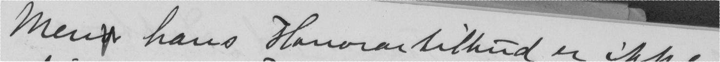Men hans Honorartilhud er ikke
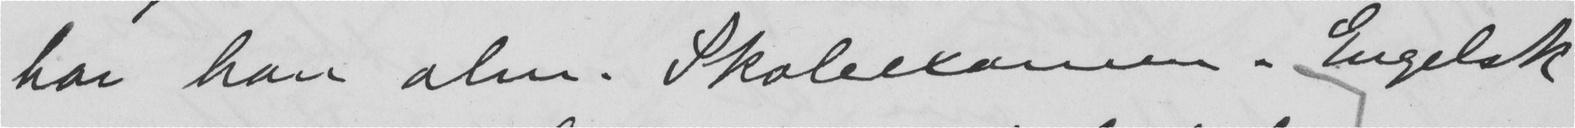har han alm. Skoleexamen. Engelsk
